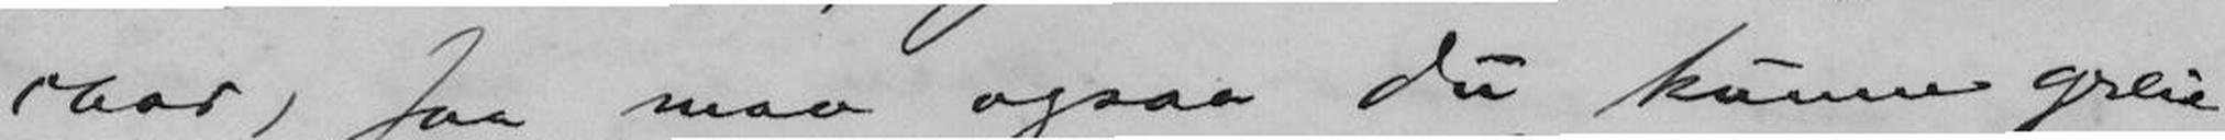iaar, saa maa ogsaa du kunne greie
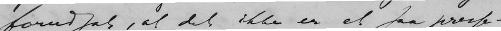forudsat, at det ikke er et saa presse-
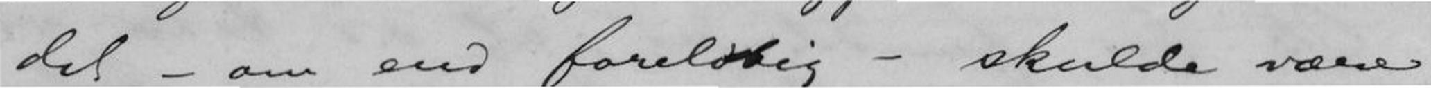det - om end foreløbig - skulde være
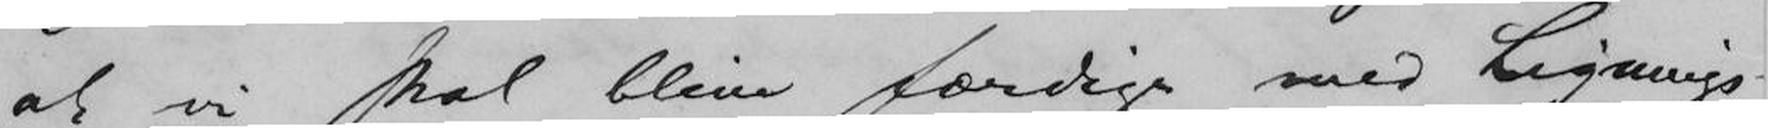at vi skal blive færdige med Lignings-
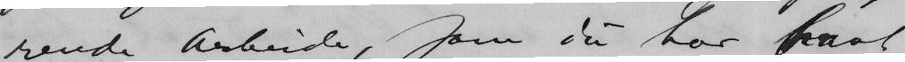rende Arbeide, som du har havt
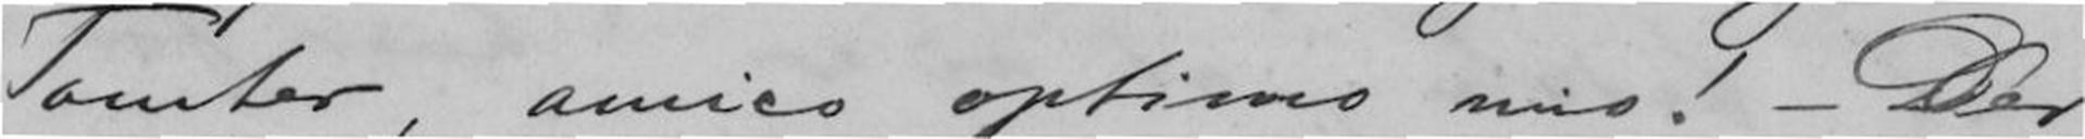Tomter, amico optimo mio! - Der
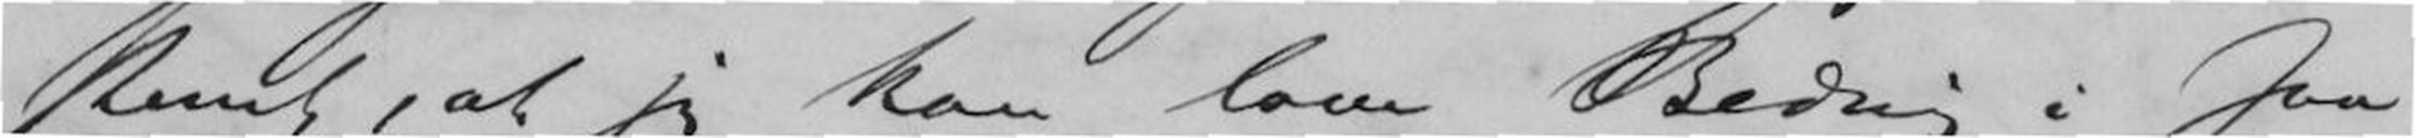stemt, at jeg kan love Bedring i saa
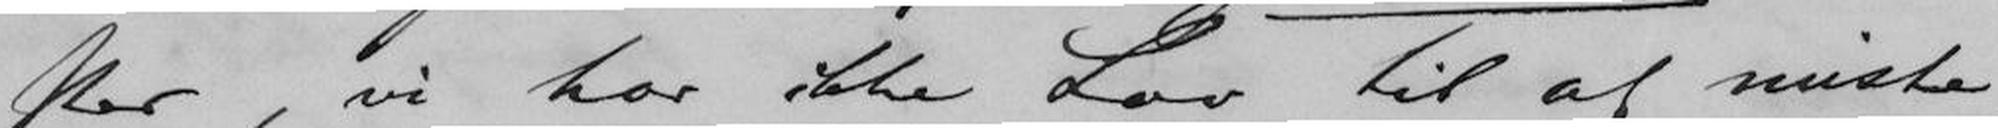ster, vi har ikke Lov til at miste
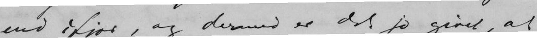end ifjor, og dermed er det jo givet, at
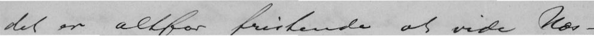det er altfor fristende at vide Næs-
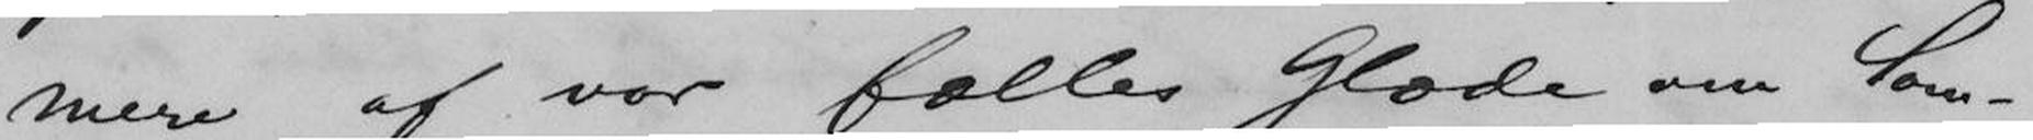mere af vor fælles Glæde om Som-
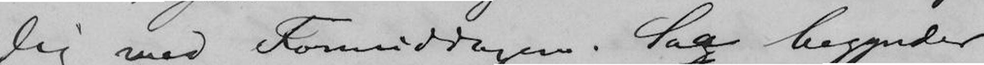dig med Formiddagen. Saa begynder
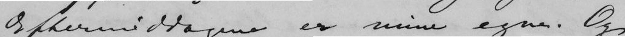Eftermiddagene er mine egne. Og
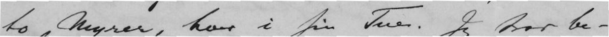to Myrer, hver i sin Tue. Jeg tror be-
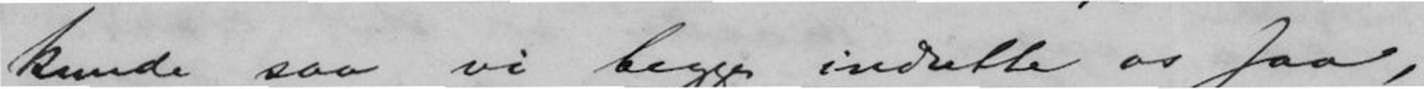kunde saa vi begge indrette os saa,
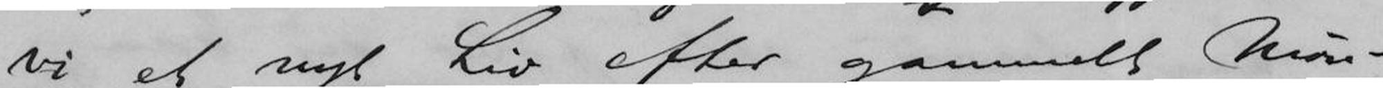vi et nyt Liv efter gammelt Møn-
